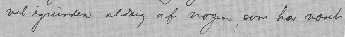vel igrunden aldrig af nogen, som har været
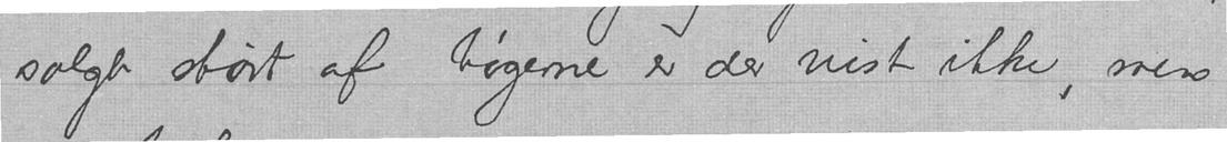solgt stort af bøgerne er der vist ikke, men
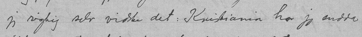jeg rigtig selv vidste det: Kristiania har jo endda
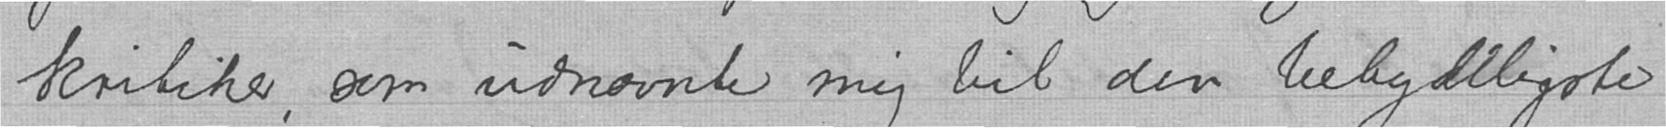kritiker, som udnævnte mig til den bebydeligste
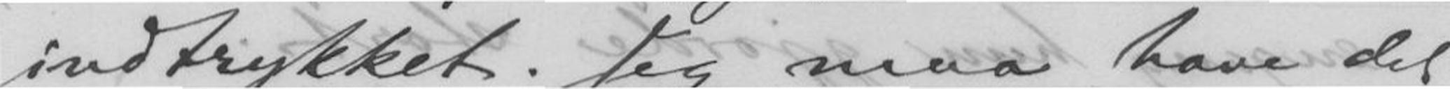indtrykket. Jeg maa have det
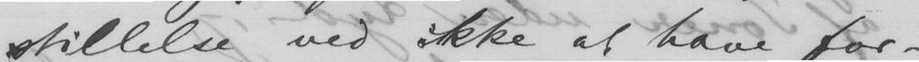stillelse ved ikke at have for-
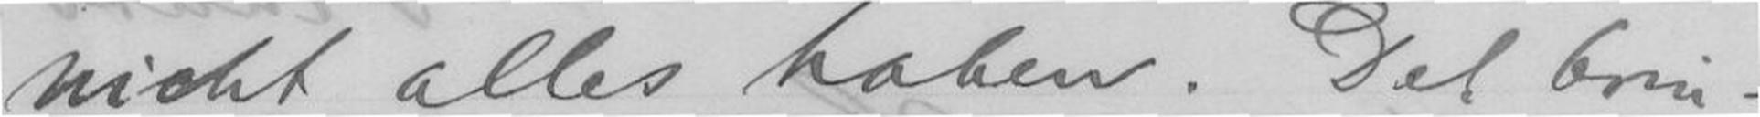nicht alles haben. Det brin-
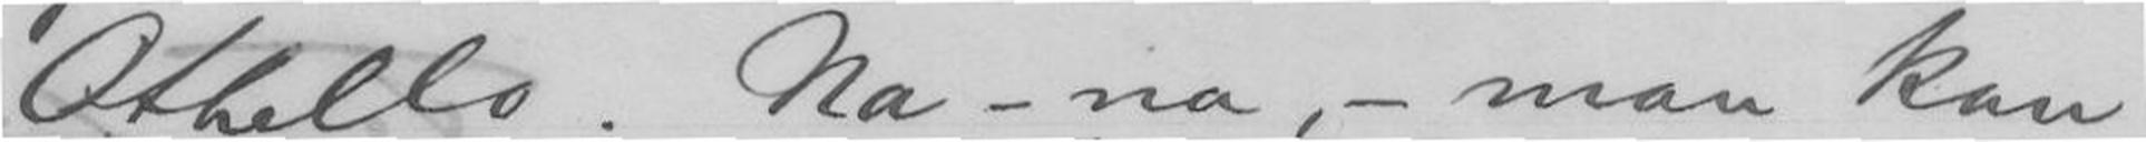Othello. Na - na, - man kan
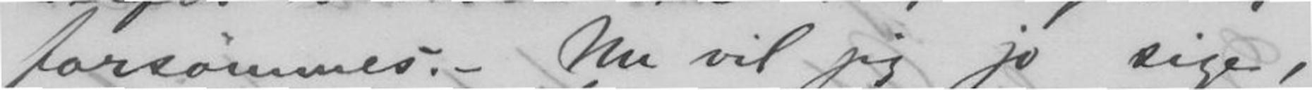forsømmes. - Nu vil jeg jo sige,
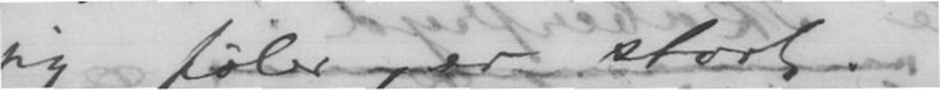jeg føler, er stort.
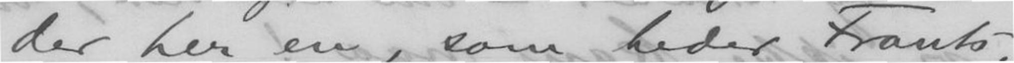der her en, som heder Frants,
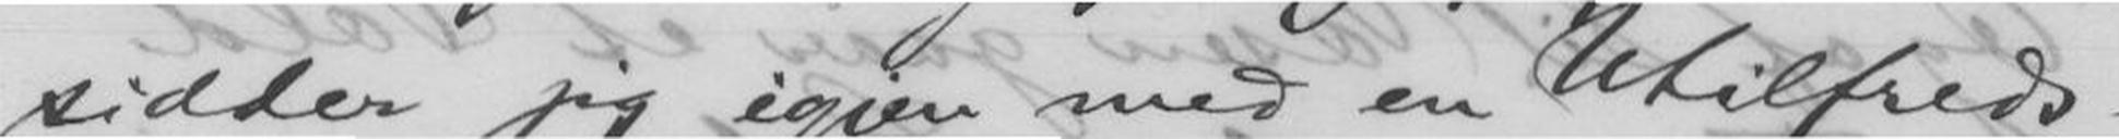sidder jeg igjen med en Utilfreds-
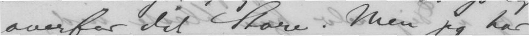overfor det Store. Men jeg har
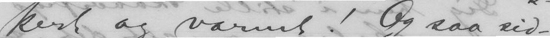kert og varmt! Og saa sid-
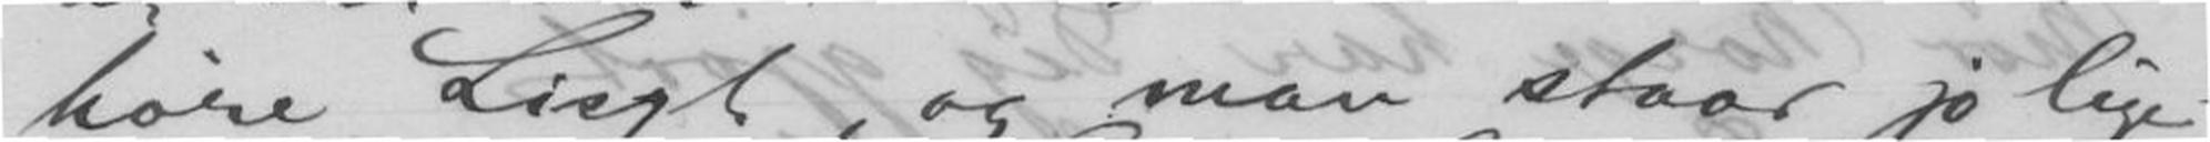høre Liszt, og man staar jo lige-
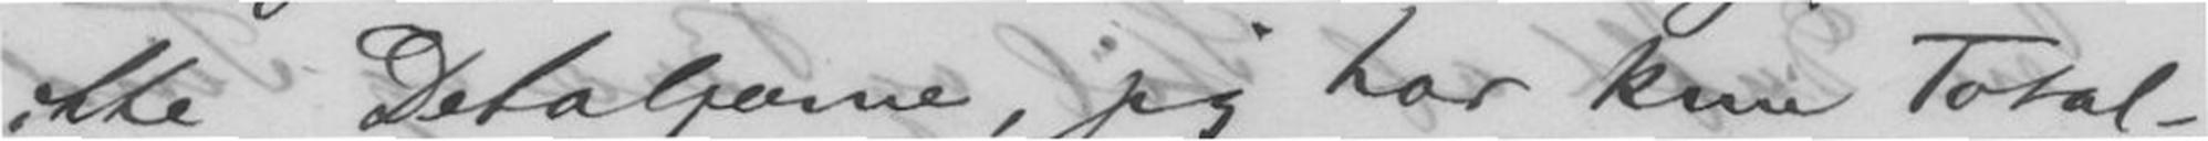ikke Detaljerne, jeg har kun Total-
